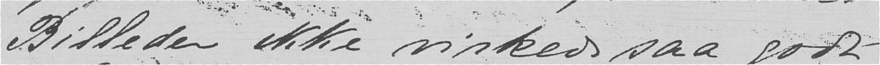Billeder ikke virkede saa godt
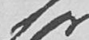for
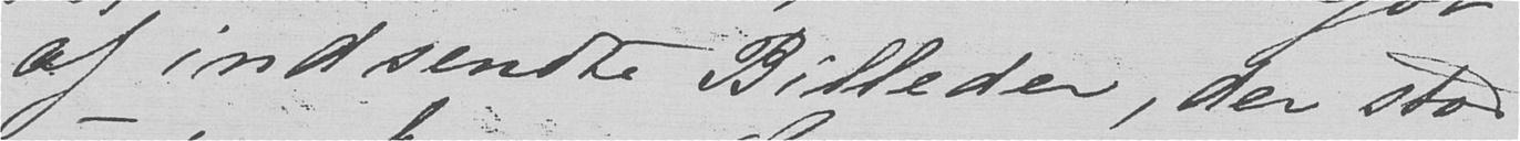af indsendte Billeder, der stod
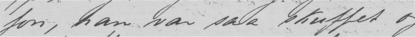fon, han var saa skuffet og
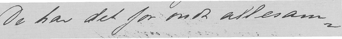De har det for ondt allesam-
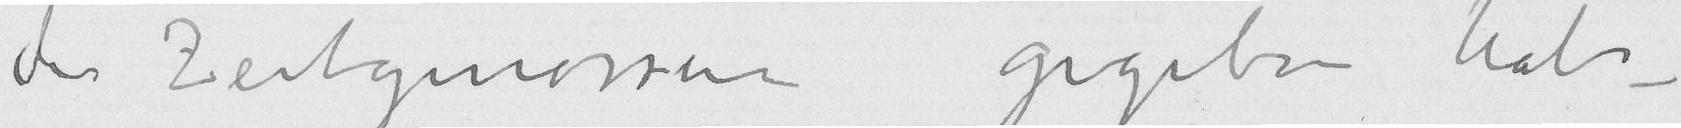der Zeitgenossen gegeben hat –
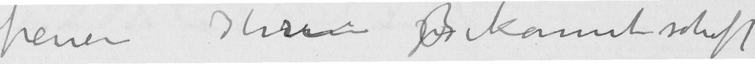freuen Ihre Bekanntschaft
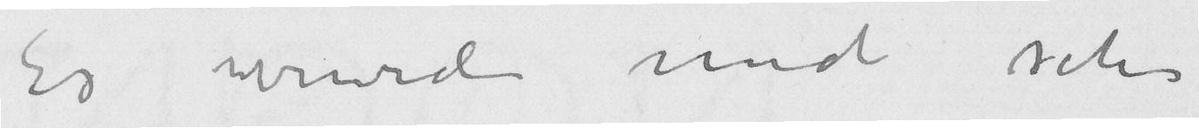Es wurde mich sehr
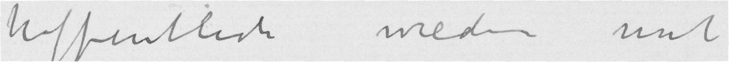hoffentlich wieder mit
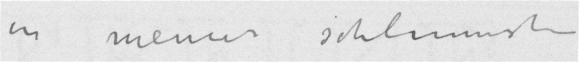in meiner schlimmsten
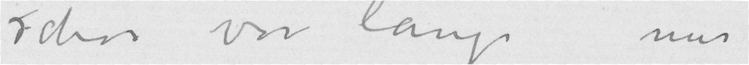schon vor lange mir
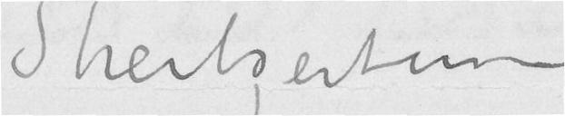Streitzeitem
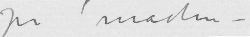zu machen –
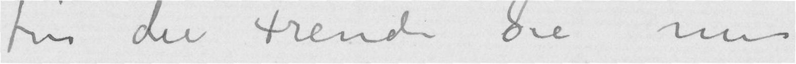für die Freude Sie mir
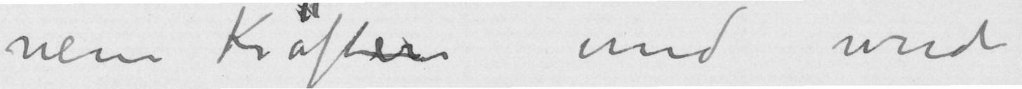neue Kräfter und wird
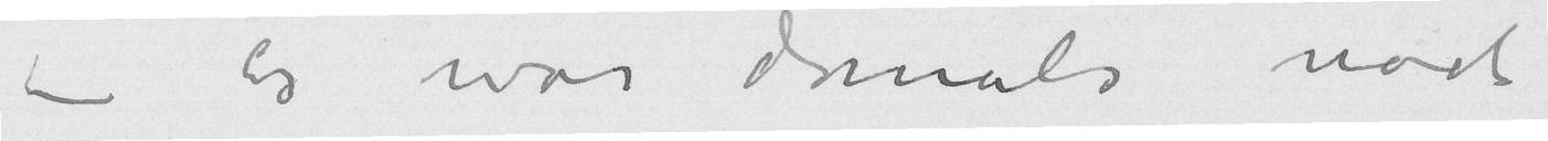– Es war damals noch
